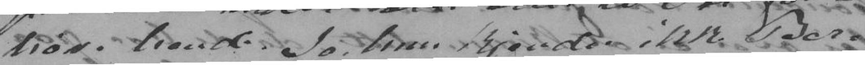høre hende. Jo, hun kjender ikke Ber-
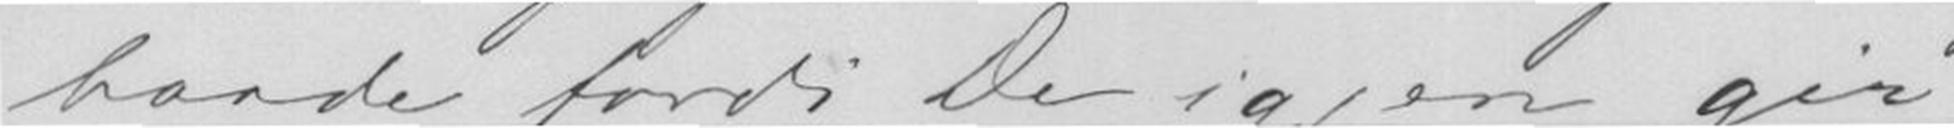baade fordi De igjen gir
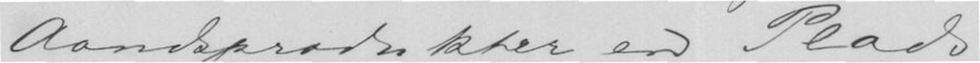Aandsprodukter en Plads
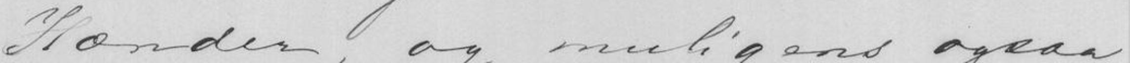Hænder, og muligens ogsaa
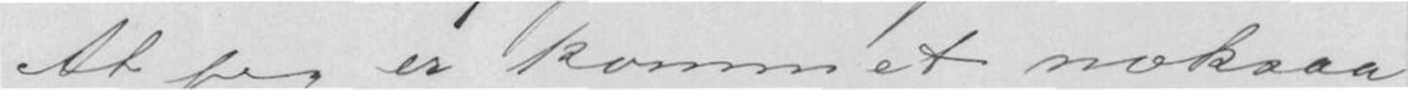At jeg er kommet noksaa
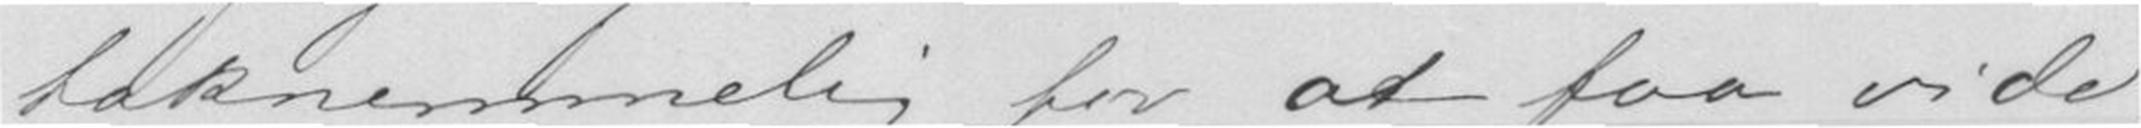taknemmelig for at faa vide.
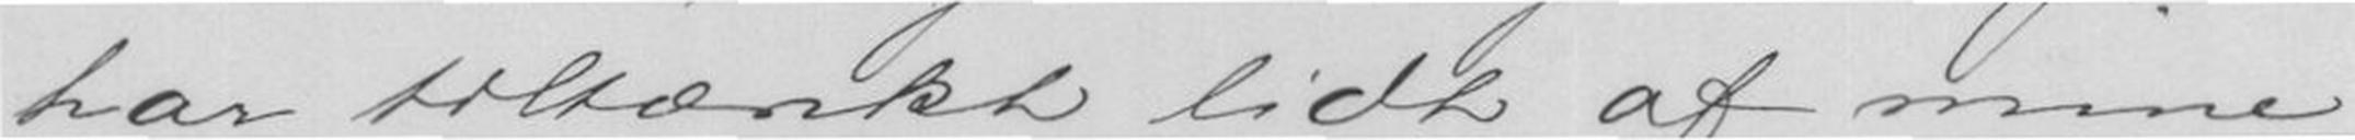har tiltænkt lidt af mine
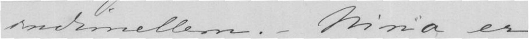indimellem.- Nina er
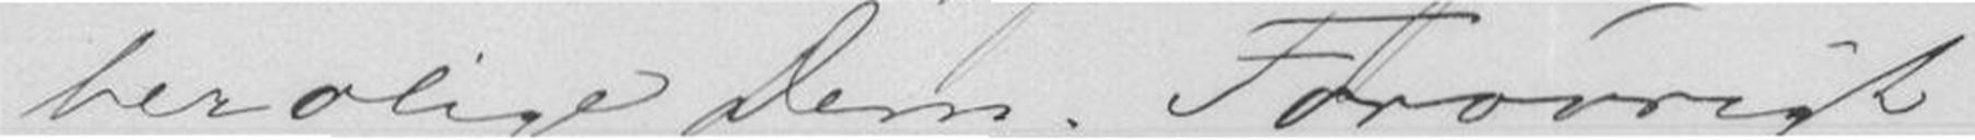berolige Dem. Forøvrigt
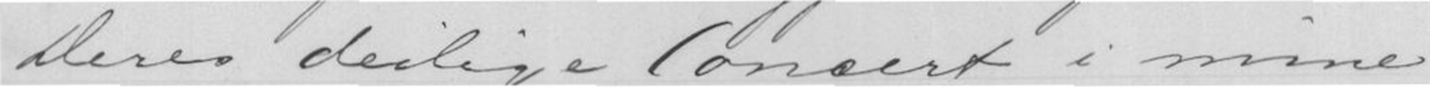Deres deilige Concert i mine
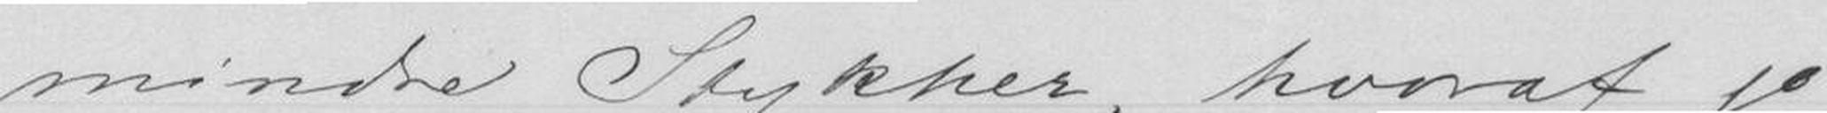mindre Stykker, hvoraf jo
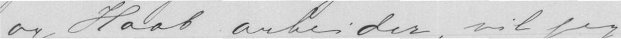og Haab arbeider, vil jeg
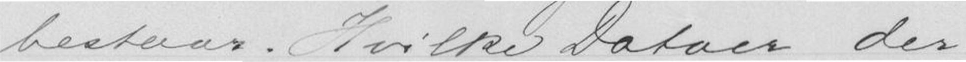bestaar. Hvilke Datoer der
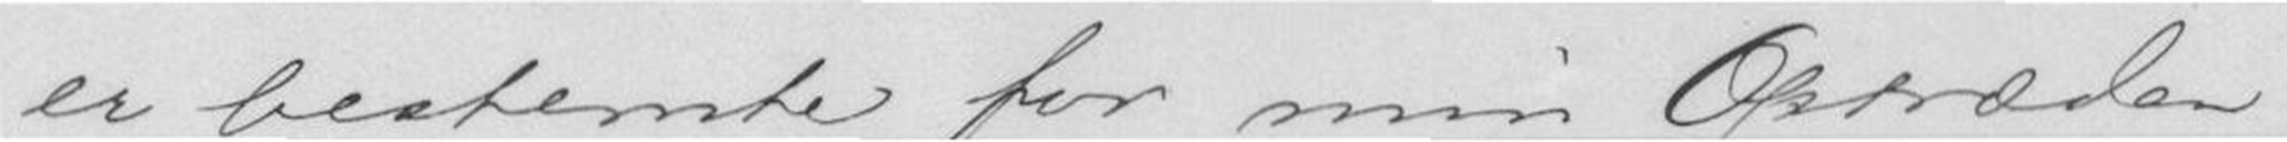er bestemte for min Optræden
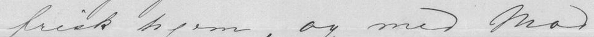frisk hjem, og med Mod
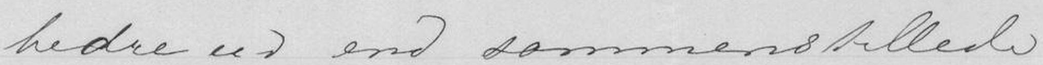bedre ud end sammenstillede
